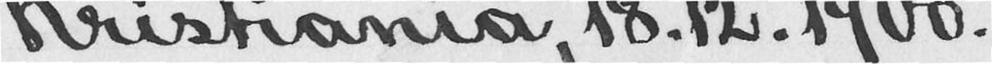Kristiania, 18.12.1900.
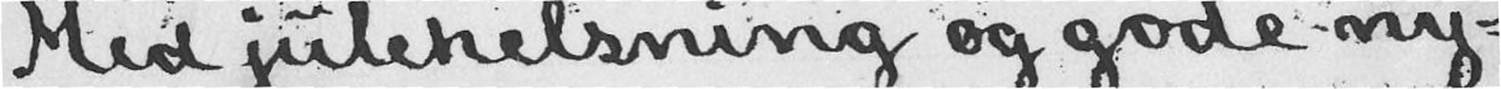Med julehelsning og gode ny-
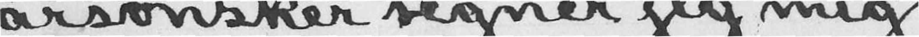årsønsker tegner jeg mig
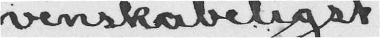venskabeligst
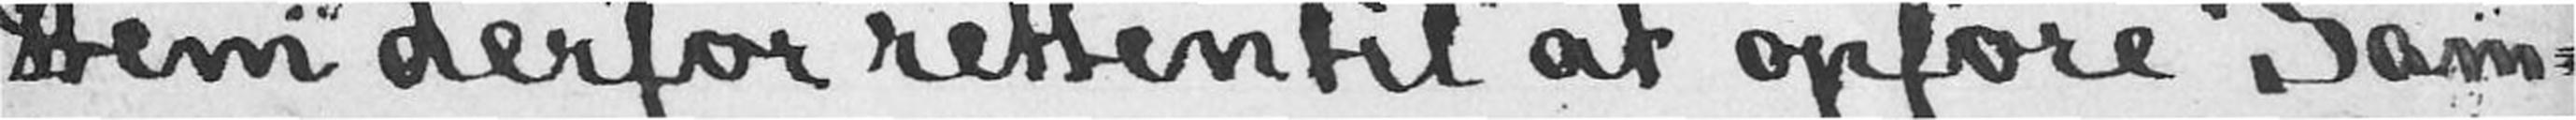Dem derfor retten til at opføre «Sam-
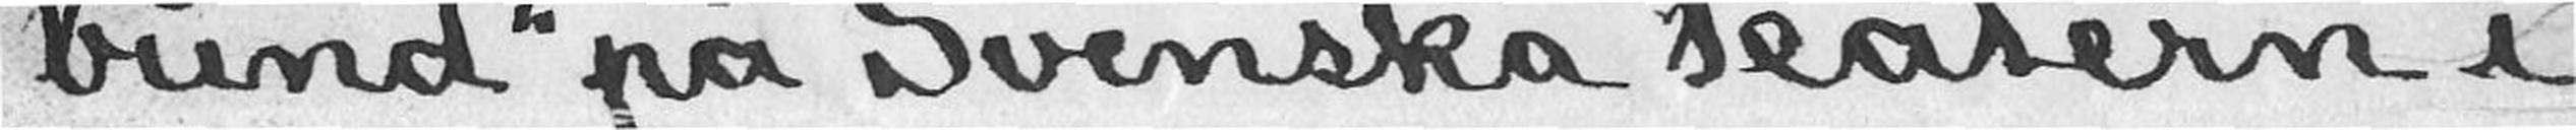bund» på Svenska Teatern i
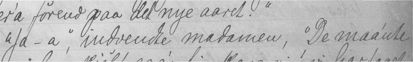"Ja - a", indvendte madamen, "De maa'nte
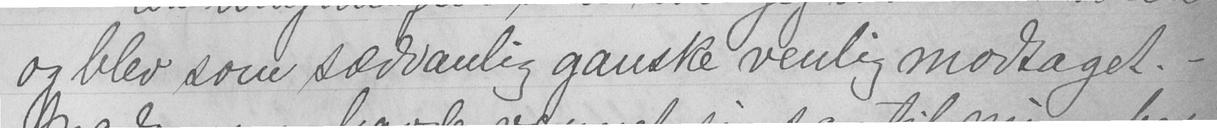og blev som sædvanlig ganske venlig modtaget. -
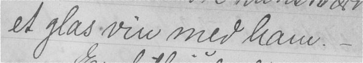et glas vin med ham. -
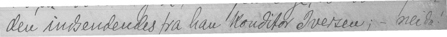den indsendendes fra han konditor Iversen; - neida!
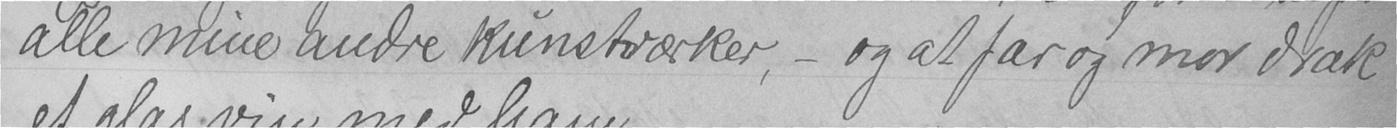alle mine andre kunstværker, - og at far og mor drak
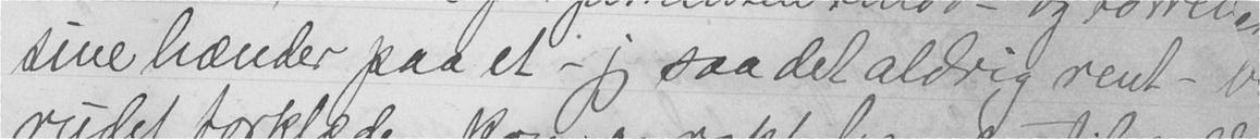sine hænder paa et - jeg saa det aldrig rent - b
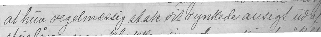at hun regelmæssig stak sit rynkede ansigt ud af
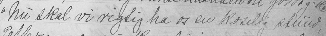"Nu skal vi rigtig ha os en koselig stund,
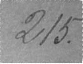215.
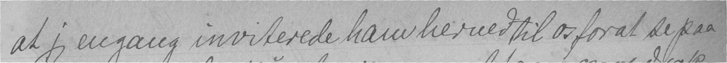at jeg engang inviterede ham herned til os forat se paa
